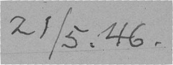21/5. 46.
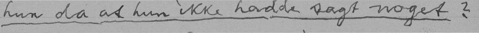hun da at hun ikke hadde sagt noget?
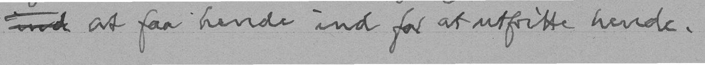ind at faa hende ind for at utfritte hende.
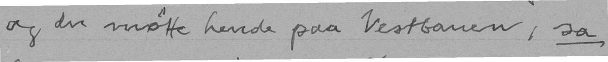og du møtte hende paa Vestbanen, sa
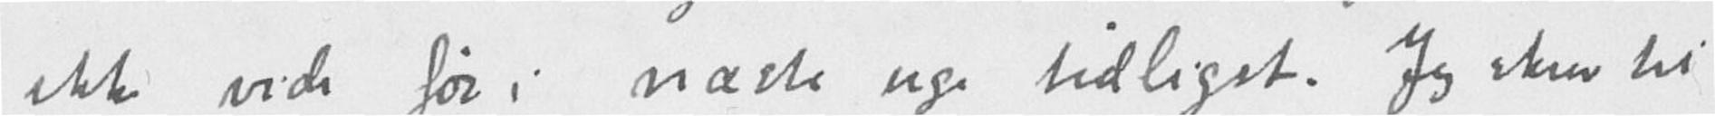ikke vide før i næste uge tidligst. Jeg skrev til
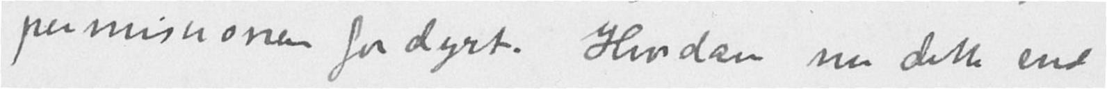permissionen for dyrt. Hvordan nu dette end
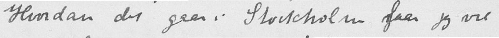Hvordan det gaar i Stockholm faar jeg vel
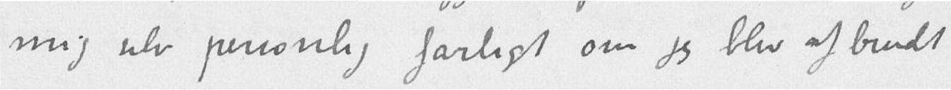mig selv personlig farligt om jeg blev afbrudt
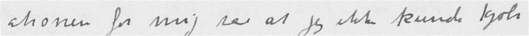ationen for mig saa at jeg ikke kunde kjøbe
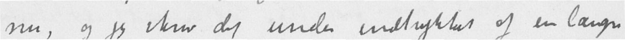nu, og jeg skrev det under indtrykket af en længre
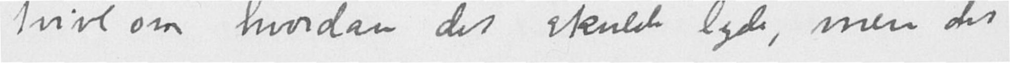tvivl om hvordan det skulde lyde, men det
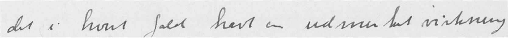det i hvert fald havt en udmerket virkning
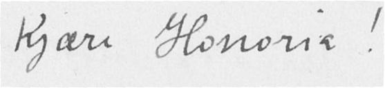Kjære Honoria!
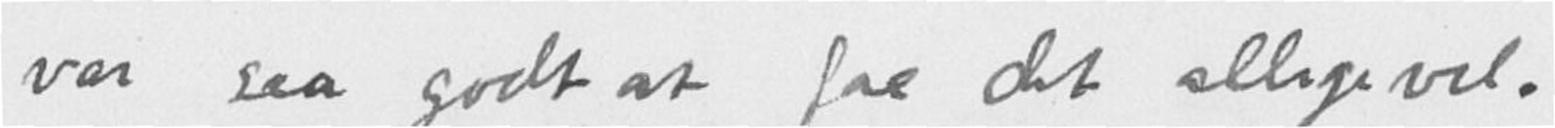var saa godt at faa det alligevel.
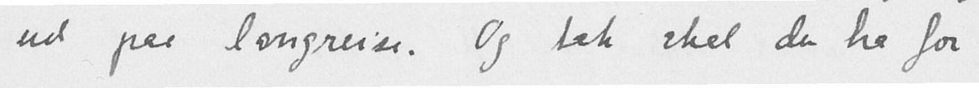ud paa langreise. Og tak skal du ha for
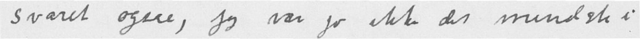svaret ogsaa, jeg var jo ikke det mindste i
